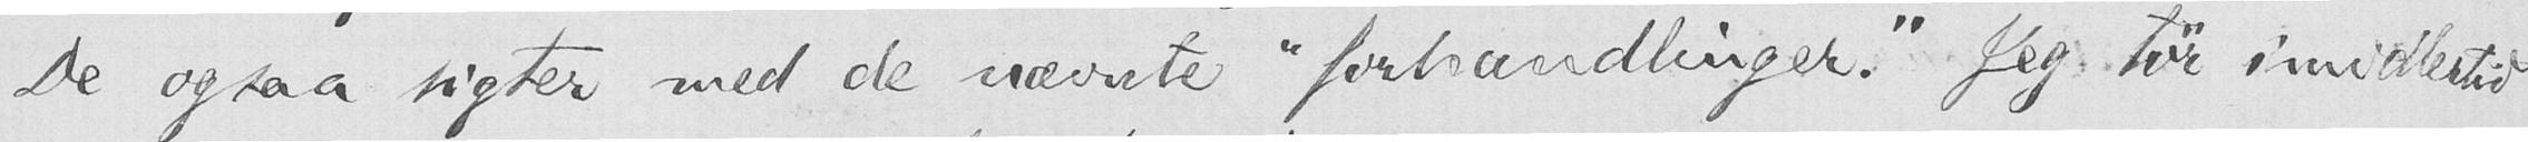De ogsaa sigter med de nævnte "forhandlinger." Jeg tør imidlertid
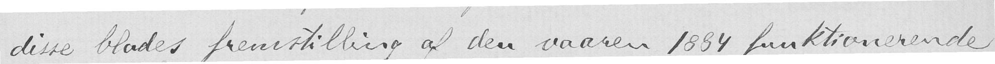disse blades fremstilling af den vaaren 1884 funktionerende
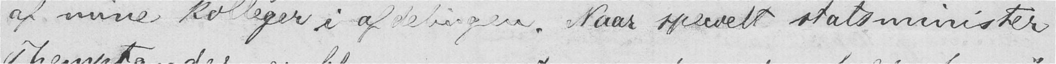af mine kolleger i afdelingen. Naar specielt statsminister
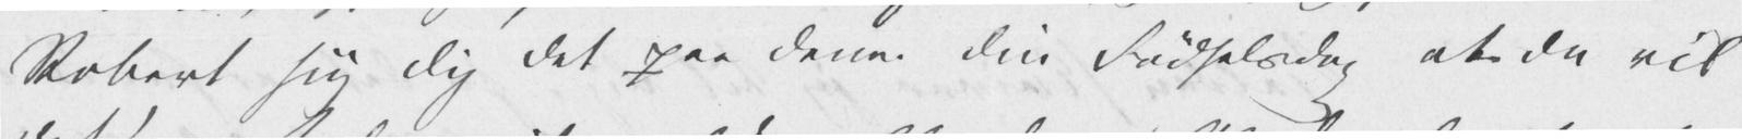Robert siig Dig det paa denne din Fødselsdag at Du vil
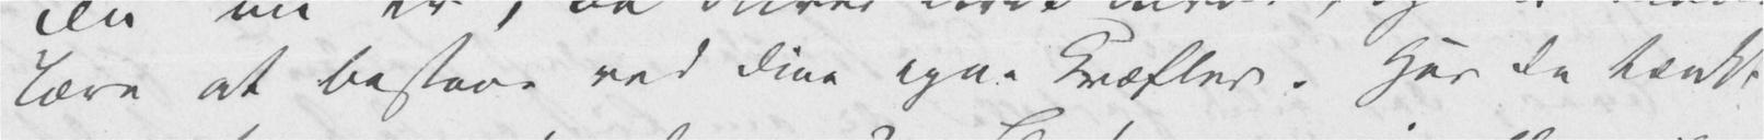lære at bestaae ved dine Egne Kræfter. Har Du tænkt
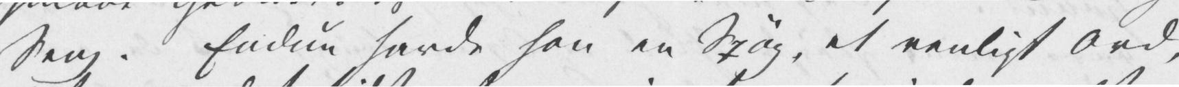Seng. Endnu havde han en Spøg, et venligt Ord,
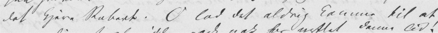det kjere Robert. O lad det aldrig komme til at
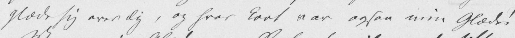glæde sig over Dig, og hvor kort var ogsaa min Glæde!
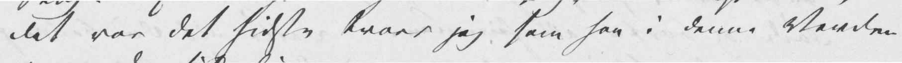det var det sidste troer jeg som han i denne Verden
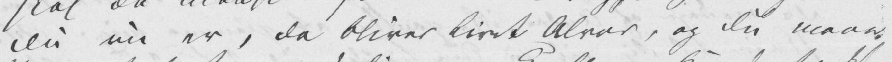Du nu er, da bliver Livet Alvor, og Du maae
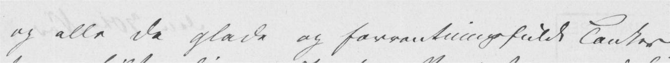og alle de glade og forventningsfulde Tanker
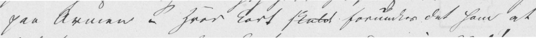paa Armen – Hvor kort skulde forundtes det ham at
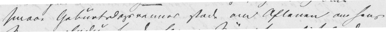smaae Geburtsdagsvenner stode om Aftenen om hans
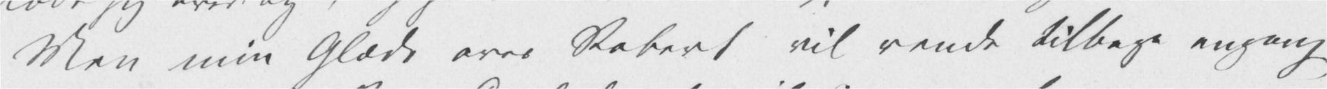Men min Glæde over Robert vil vende tilbage engang
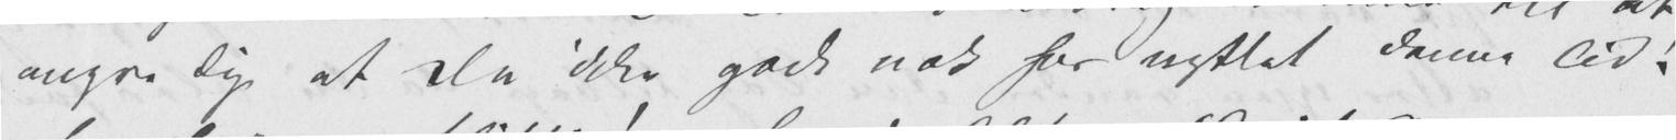angre Dig at Du ikke godt nok har nyttet denne Tid!
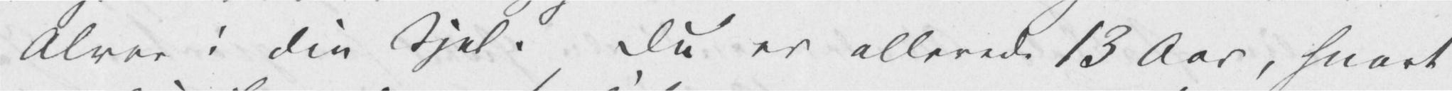Alvor i Din Sjel. Du er allerede 13 Aar, snart
